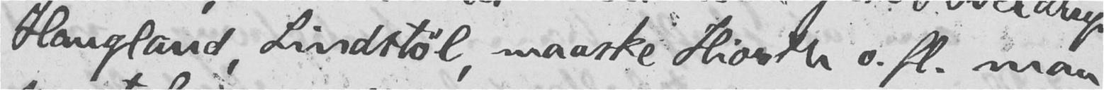Haugland, Lindstøl, maaske Hiorth o. fl. maa
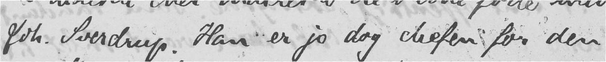Joh. Sverdrup. Han er jo dog chefen for den
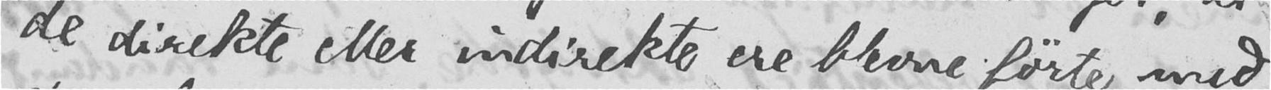de direkte eller indirekte ere blevne førte med
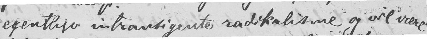egentlige intransigente radikalisme og vil være
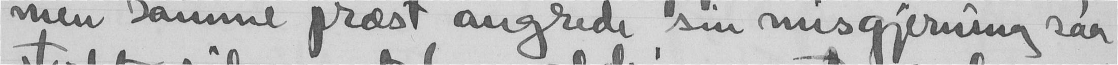men samme præst angrede sin misgjerning saa
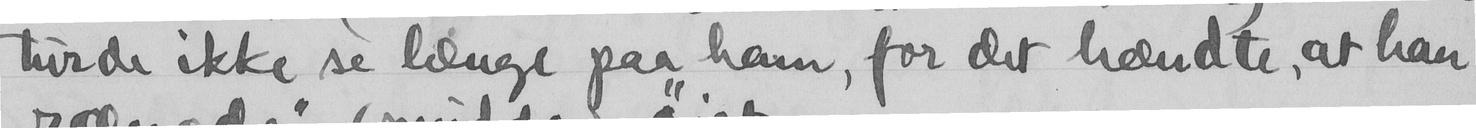turde ikke se længe paa ham, for det hændte, at han
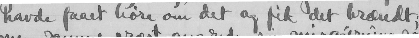havde faaet høre om det og fik det brændt;
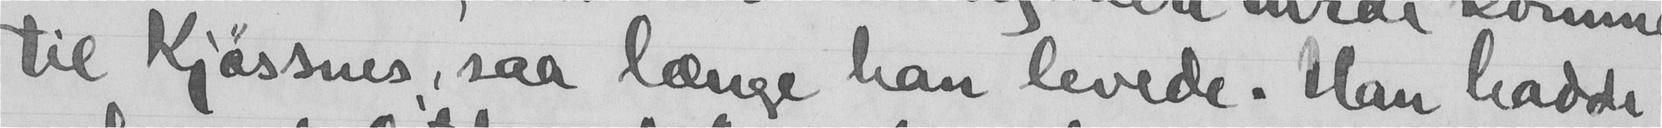til Kjøssnes, saa længe han levede. Han hadde
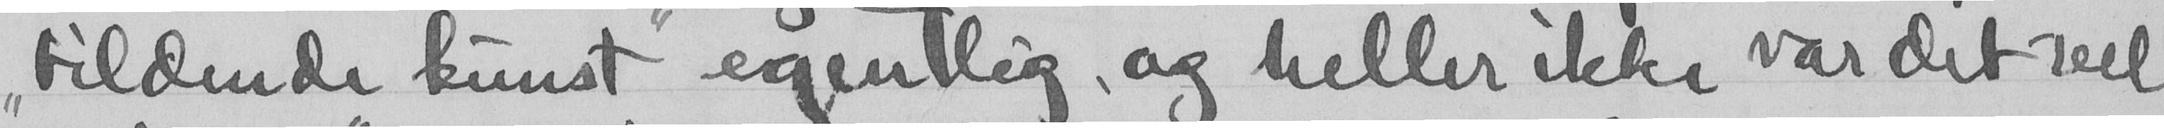"bildende kunst" egentlig, og heller ikke var det vel
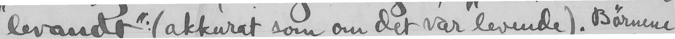levandt": (akkurat som om det var levende). Børnene
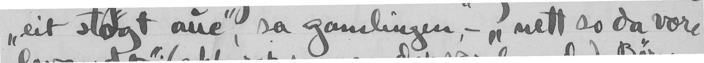"eit støgt aue", sa gamlingen, - "nett so da være
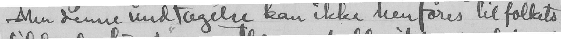Men denne undtagelse kan ikke henføres til folkets
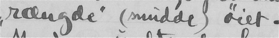"rængde" (snudde) øiet.
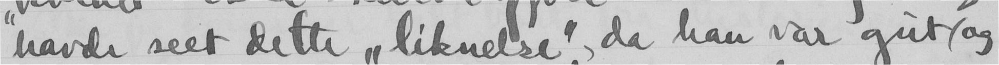havde seet dette "liknelse", da han var gut og
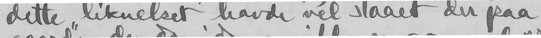dette "liknelset" havde vel staaet der paa
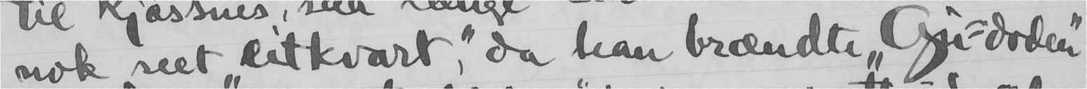nok seet "eitkvart"; da han brændte "Gu-doden"
